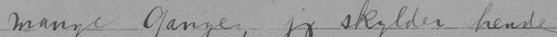mange Gange, jeg skylder hende
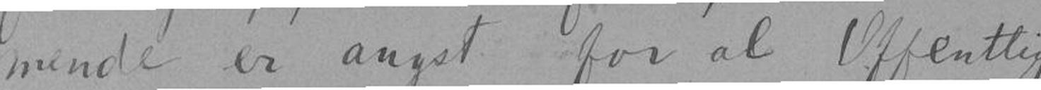mende er angst for al Offentlig
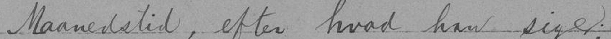Maanedstid, efter hvad han siger;
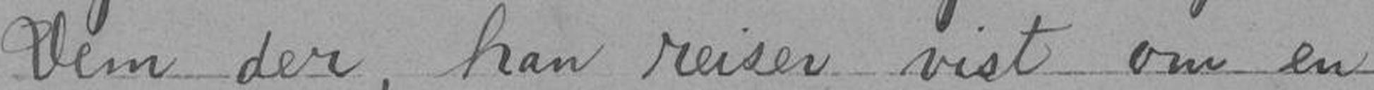Dem der, han reiser vist om en
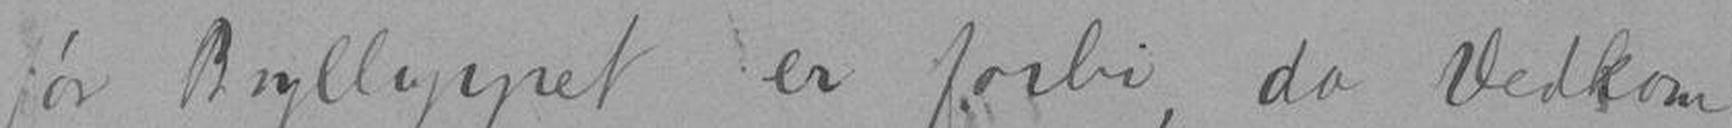før Brylluppet er forbi, da Vedkom
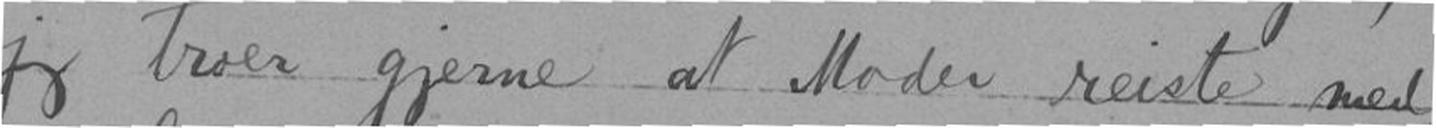jeg troer gjerne at Moder reiste med,
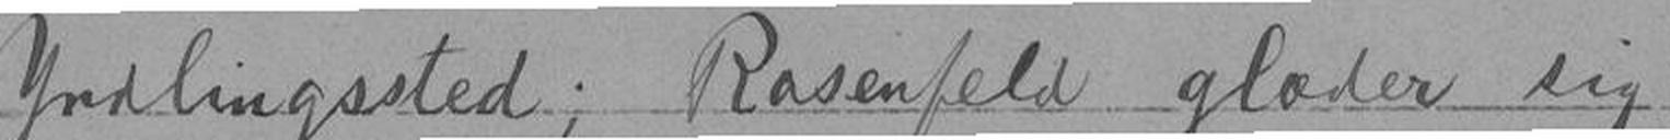Yndlingssted; Rasenfeld glæder sig
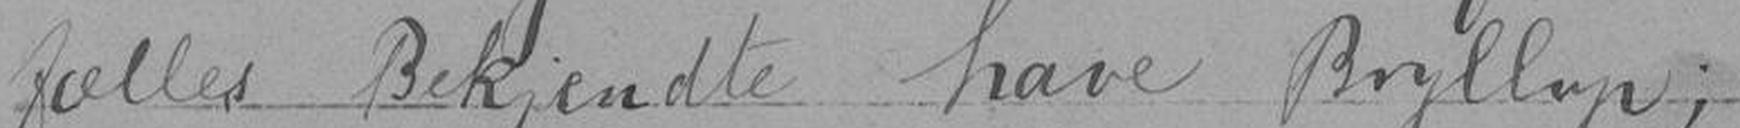fælles Bekjendte have Bryllup;
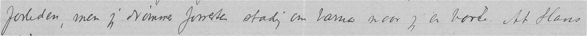forleden, men jeg drømmer forresten stadig om barna naar jeg er borte. At Hans
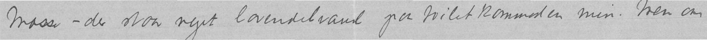Mosse - der staar noget lavendelvand paa toiletkommoden min. Men om
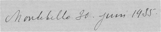Montebello 30. juni 1935.
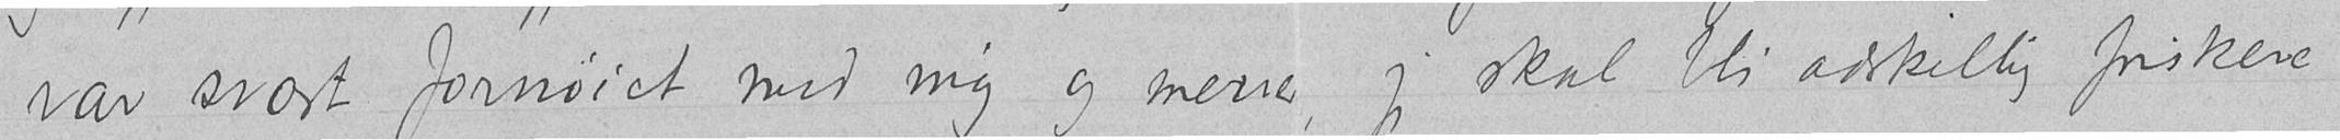var svært fornøiet med mig og mener, jeg skal bli adskillig friskere
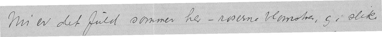Nu er det fuld sommer her – roserne blomstrer, og i slike
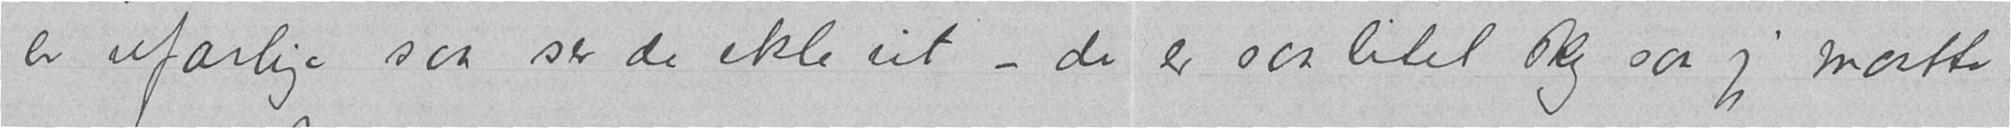er ufarlige saa ser de ekle ut – de er saa litet sky saa jeg maatte
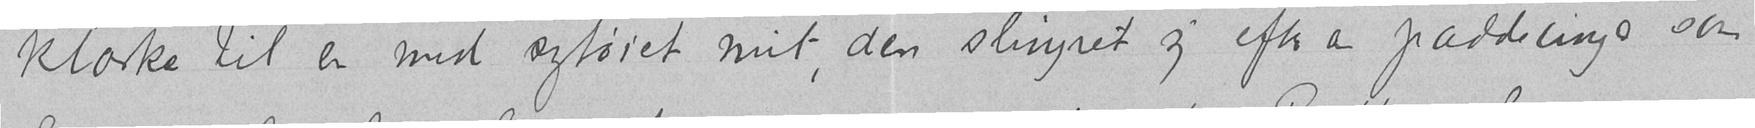klaske til en med sytøiet mit, den slingret sig efter en paddelunge som
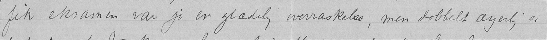fik eksamen var jo en glædelig overraskelse, men dobbelt ærgerlig er
